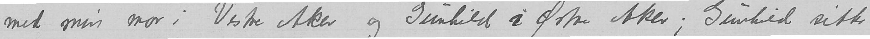med min mor i Vestre Aker og Gunhild i Østre Aker; Gunhild sitter
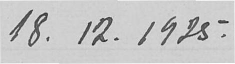18.12.1925.
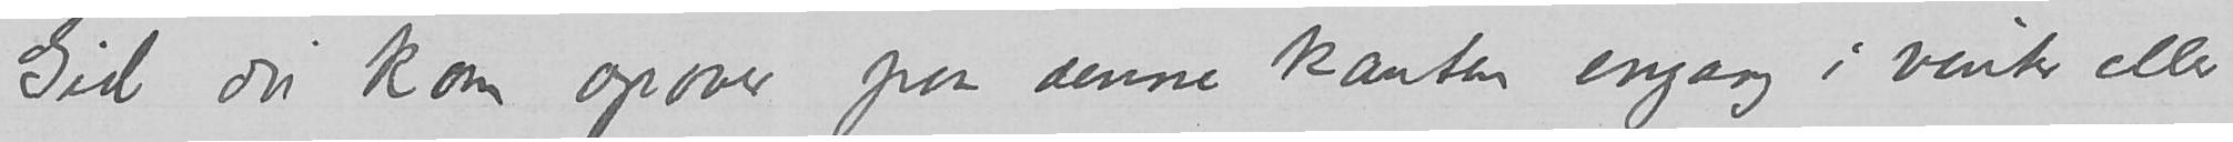Gid du kom opover paa denne kanten engang i vinter eller
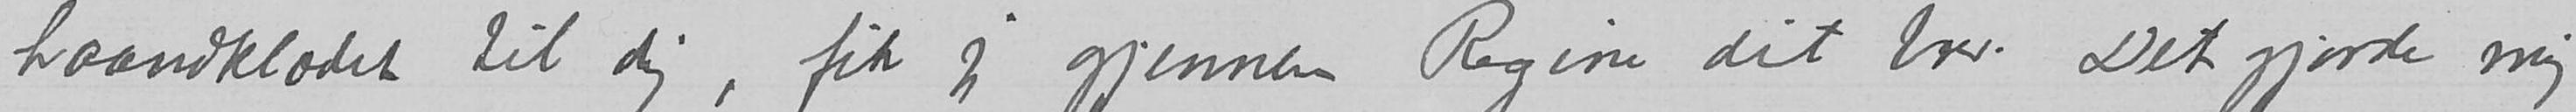haandklædet til dig, fik jeg gjennem Regine dit brev. Det gjorde mig
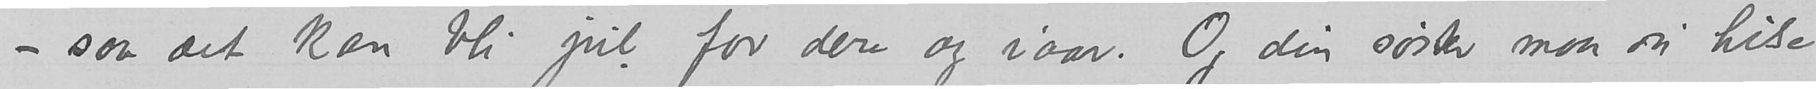– saa det kan bli jul for dere og iaar. Og din søster maa du hilse
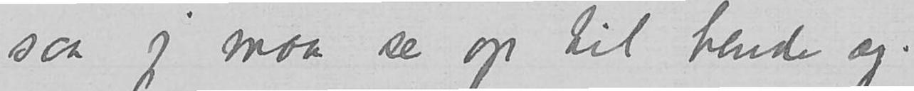saa jeg maa se op til hende og.
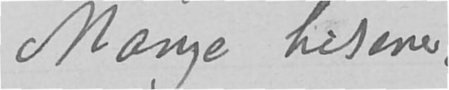Mange hilsener,
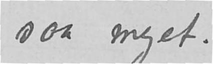saa meget.
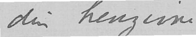din hengivne
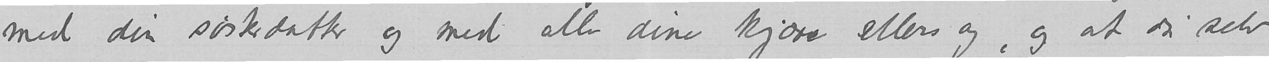med din søsterdatter og med alle dine kjære ellers og, og at du selv
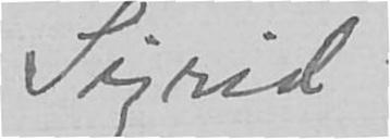Sigrid.
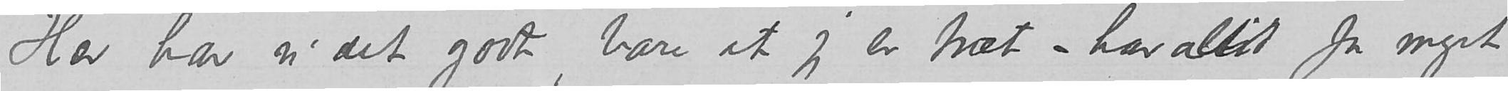Her har vi det godt, bare at jeg er træt – har altid for meget
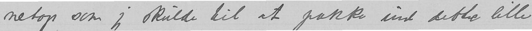netop som jeg skulde til at pakke ind dette lille
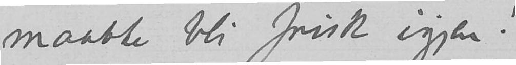maatte bli frisk igjen!
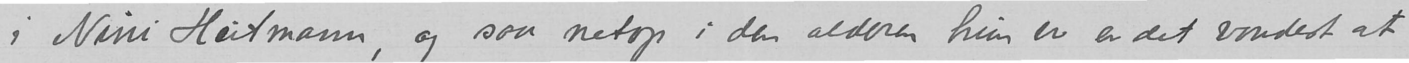i Nini Heitmann, og saa netop i den alderen hun er er det vondest at
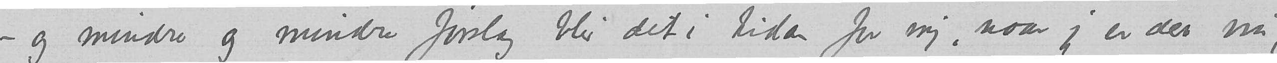– og mindre og mindre <forslag> blir det i tiden for mig, naar jeg er der nu,
